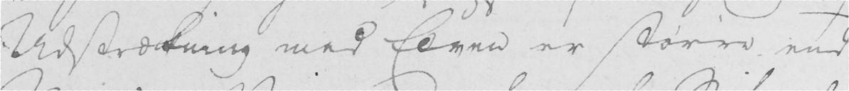Udstrækning med Elven er større end
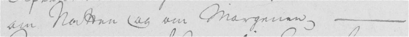om Natten og om Morgenen -
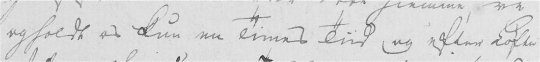opholdt os kun en Times Tiid og efter Løfte
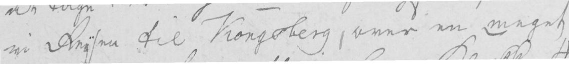vi Reysen til Kongsberg, over en meget
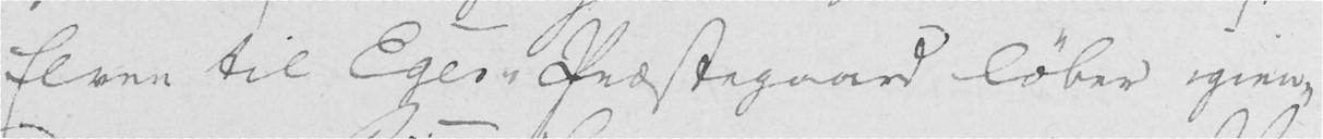Elven til Eger Præstegaard løber igien-
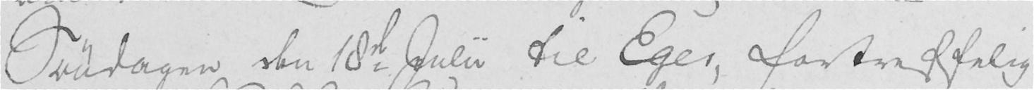Søndagen den 18de Julii til Eger, fortreffelig
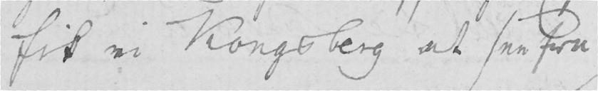fik vi Kongsberg at see fra
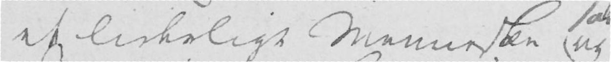et liderligt Menneske og
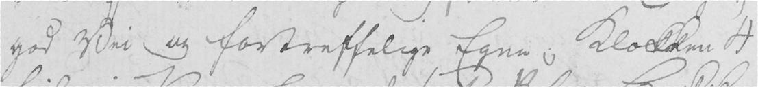god Vei og fortreffelige Egne; Klokken 4
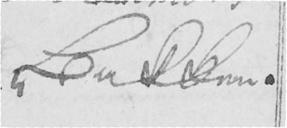Bakken.
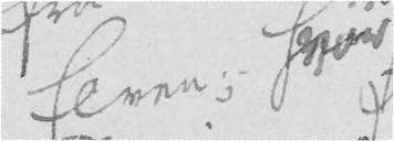Elven; hvor
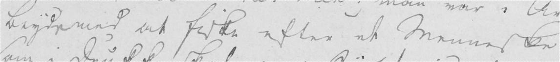beyde med at fiske efter et Menneske
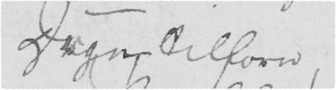Dage tilforn
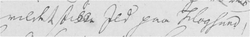vildet stikke Ild paa Hogsund,
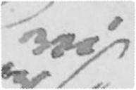vi
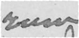ram
